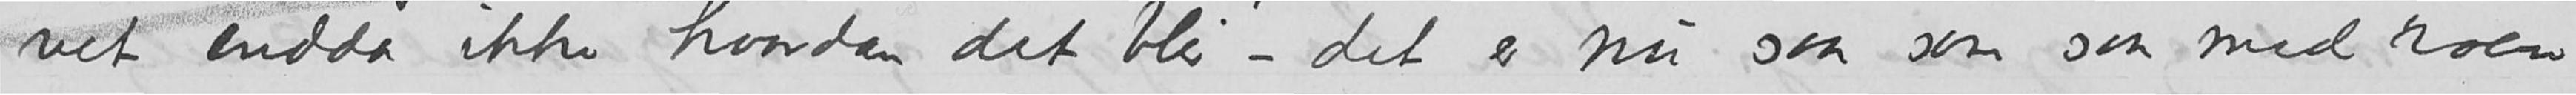vet endda ikke hvordan det blir – det er nu saa som saa med roen
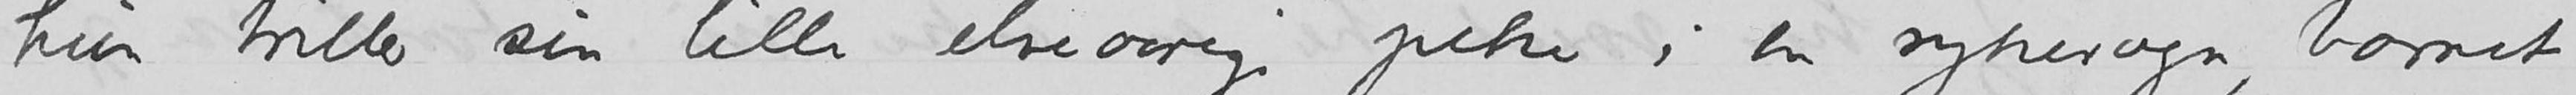hun triller sin lille elveaarige pike i en sykevogn, barnet
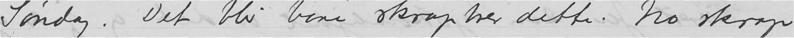Søndag. Det blir bare skrapbrev dette. No skrap
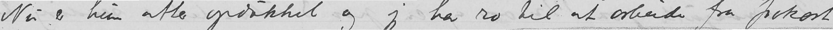Nu er hun atter opdukket og jeg har ro til at arbeide fra frokost
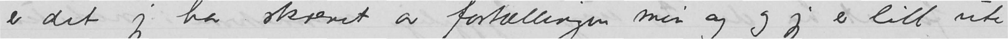er det jeg har skrevet av fortællingen min og og jeg er litt ute
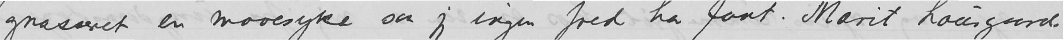spasseret en mavesyke saa jeg ingen fred har faat. Marit Laurgaard
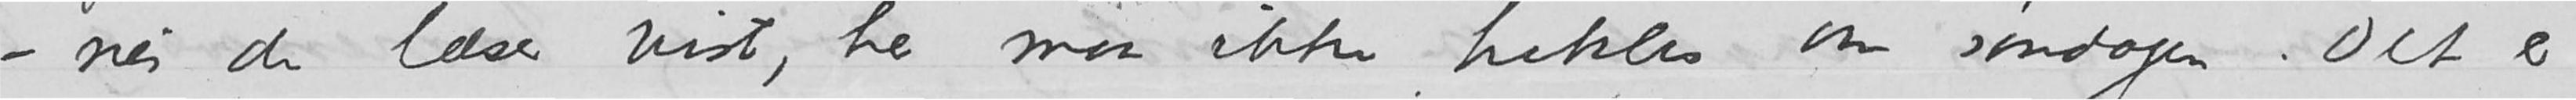– nei de læser vist, her maa ikke hekles om søndagen. Det er
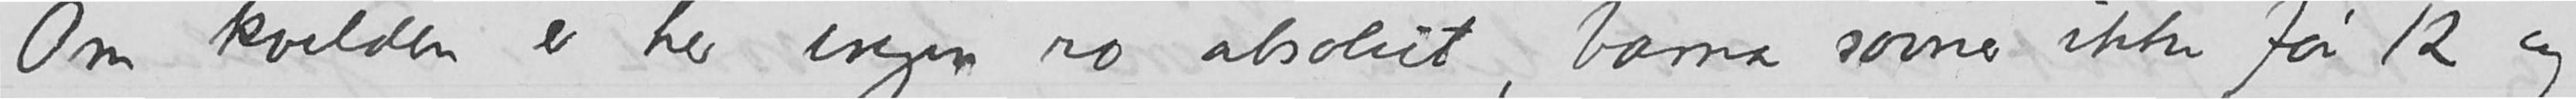. Om kvelden er her ingen ro absolut, barna sovner ikke før 12 og
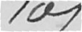10
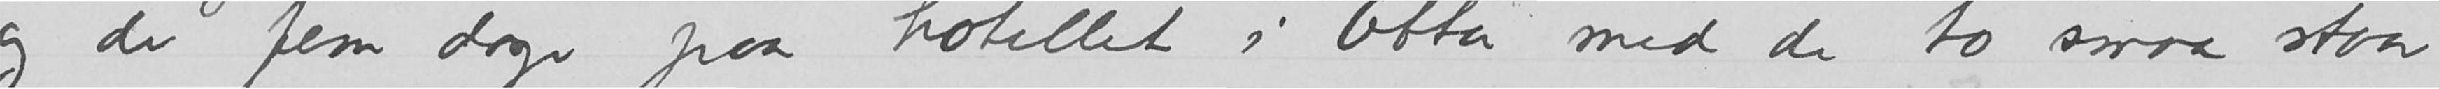og de fem dage paa hotellet i Otta med de to smaa staar
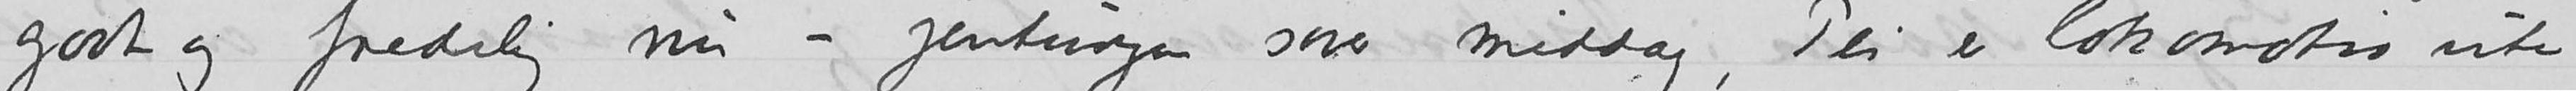godt og fredelig nu – jentungen sover middag, Pei er lokomotiv ute
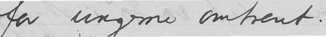for ungerne omtrent.
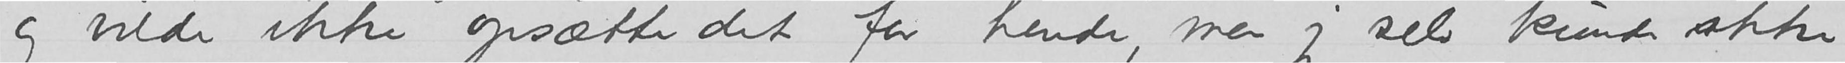og vilde ikke opsætte det for hende, men jeg selv kunde ikke
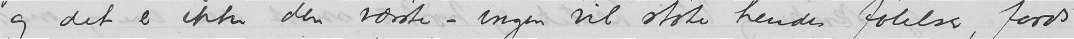og det er ikke den værste – ingen vil støte hendes følelser, fordi
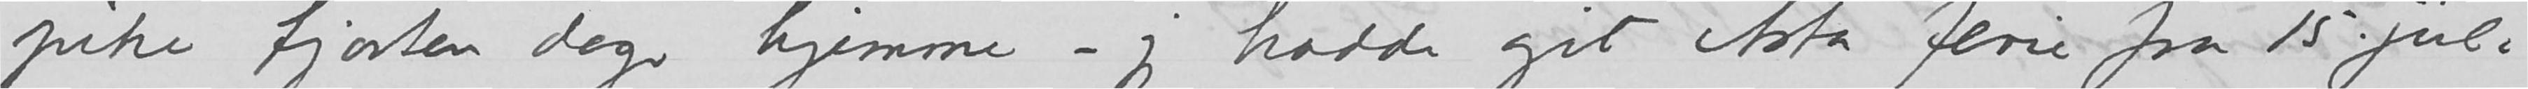pike fjorten dage hjemme – jeg hadde git Asta ferie fra 15. juli
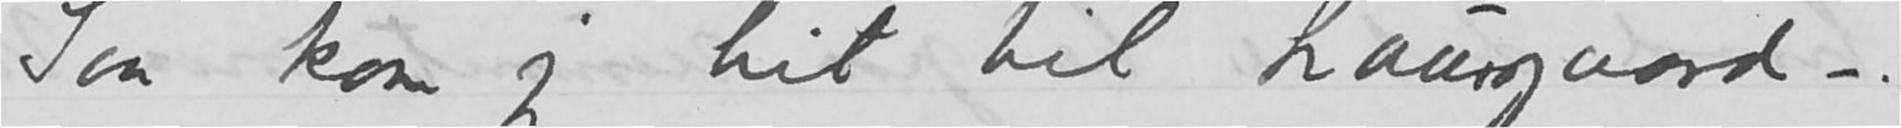Saa kom jeg hit til Laurgaard. -.
